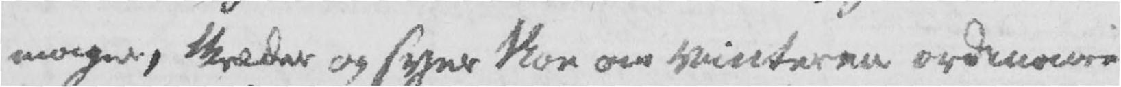mager, Skræder og syer Skoe om Vinteren ordinaire
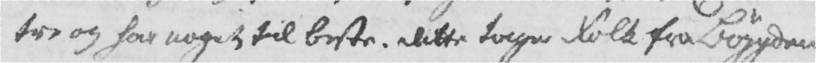tre og har noget til beste. Dette tager Folk fra Bøygden
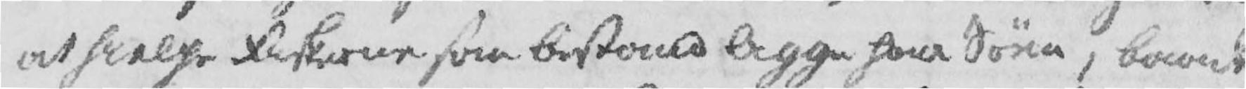at hielpe Fiskerne som bestand ligge paa Søen, baade
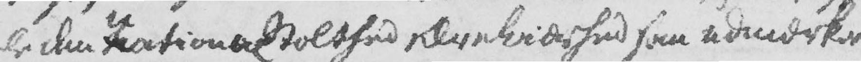le den Nationalstolthed Ærekiærhed som udmærker
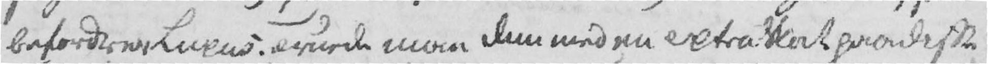befordrer Luxus. Truede man dem med en Extra Skat paa disse
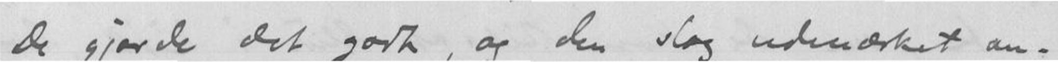De gjorde det godt, og den slog udmærket an.
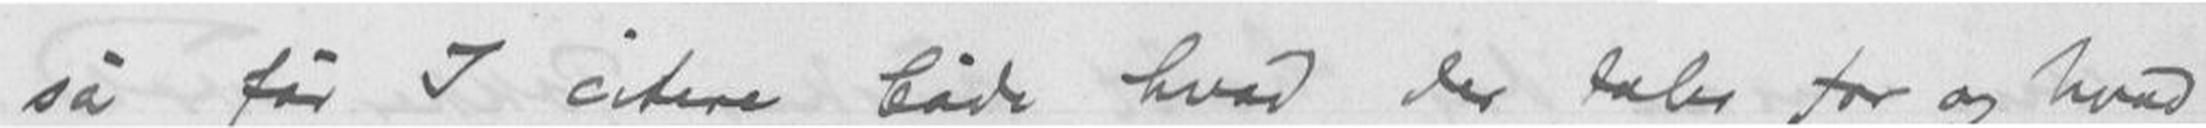så får I citere både hvad der taler for og hvad
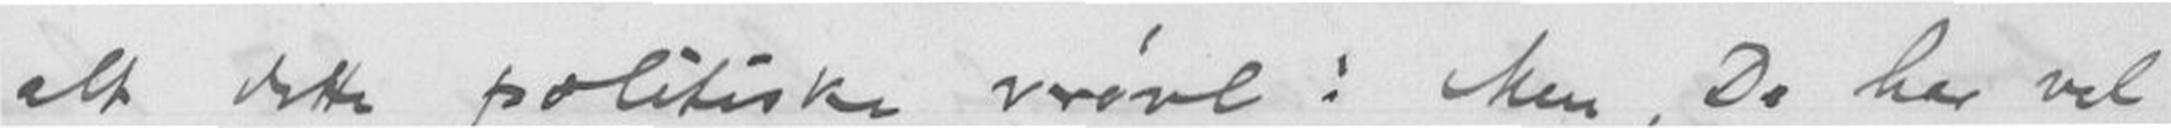alt dette politiske vrøvl: Men, de har vel
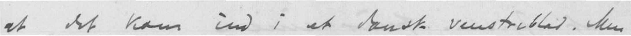at det kom ind i et dansk venstreblad. Men
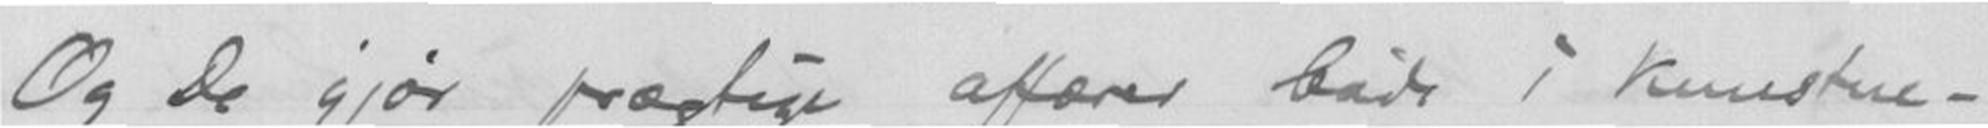Og De gjør prægtige affærer både i kunstne-
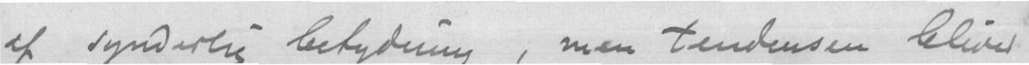af synderlig betydning, men tendensen bliver
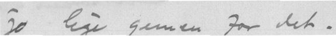jo lige gemen for det.
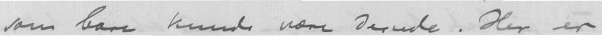som bare kunde være derude. Her er
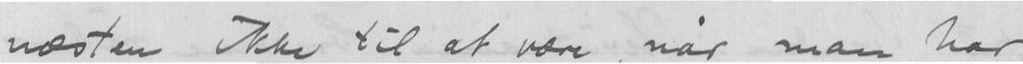næsten ikke til at være, når man har
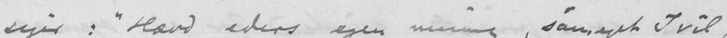siger: "hvad eders egen mening, såmeget I vil,
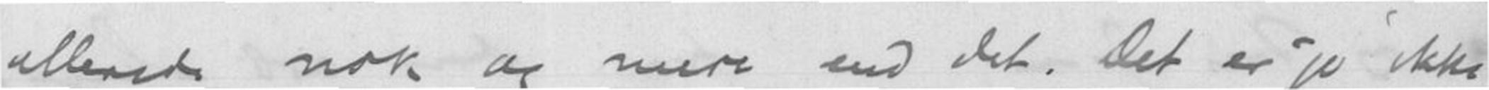allerede nok og mere end det. Det er jo ikke
